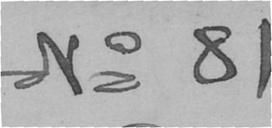No 81
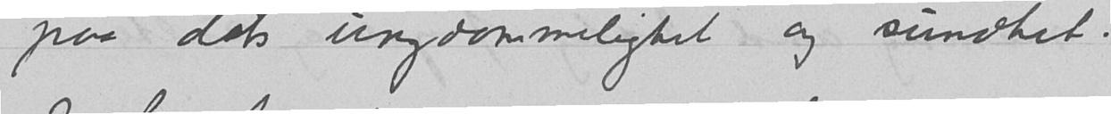paa dets ungdommelighet og sundhet.
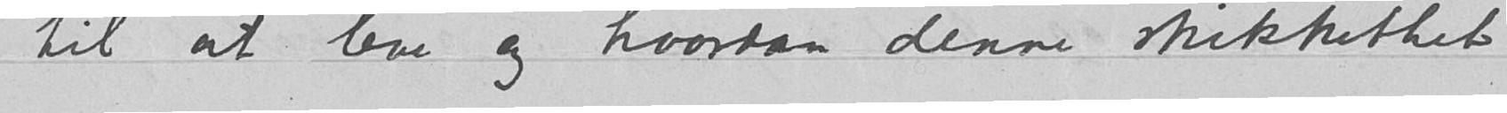til at leve og hvordan denne skikkethet
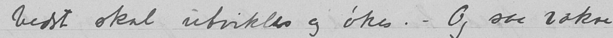bedst skal utvikles og økes. - Og saa vakre
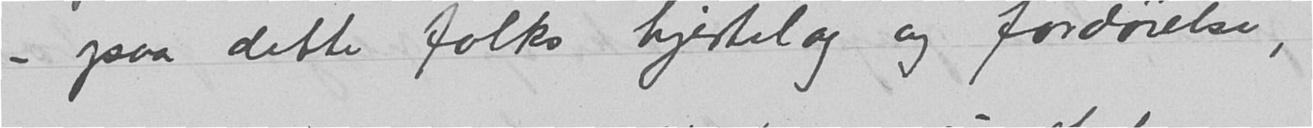- paa dette folks hjertelag og fordøielse,
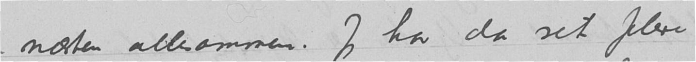- næsten allesammen. Jeg har da set flere
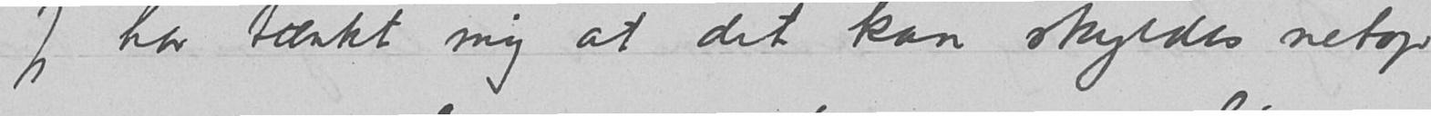Jeg har tænkt mig at det kan skyldes netop
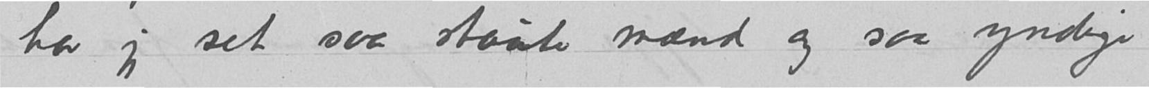har jeg set saa staute mænd og saa yndige
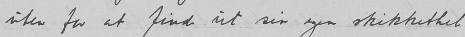uten for at finde ut sin egen skikkethet
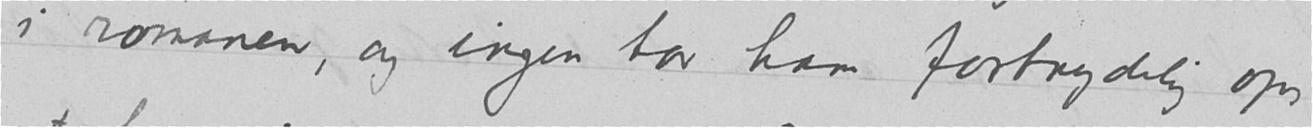i romanen, og ingen tar han fortrydelig op
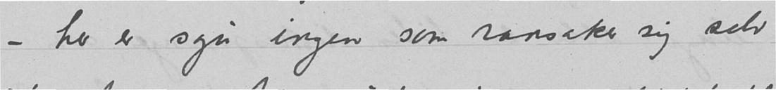– her er sgu ingen som ransaker sig selv
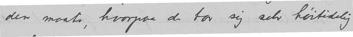den maate, hvorpaa de tar sig selv høitidelig
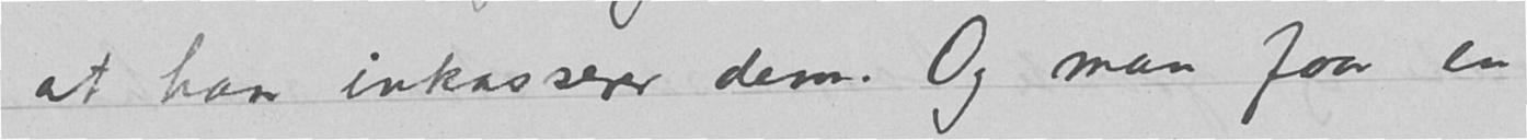at han inkasserer dem. Og man faar en
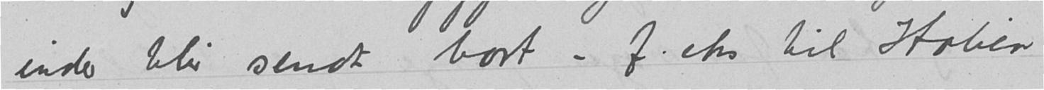inder blir sendt bort – f.eks. til Italien
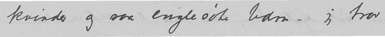kvinder og saa englesøte barn - jeg tror
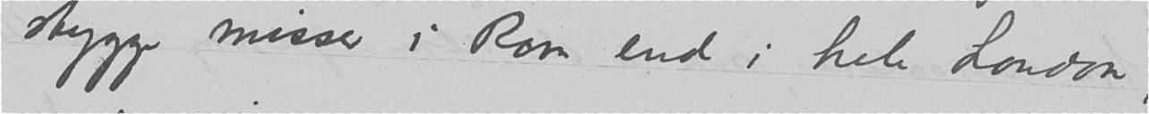stygge misser i Rom end i hele London,
